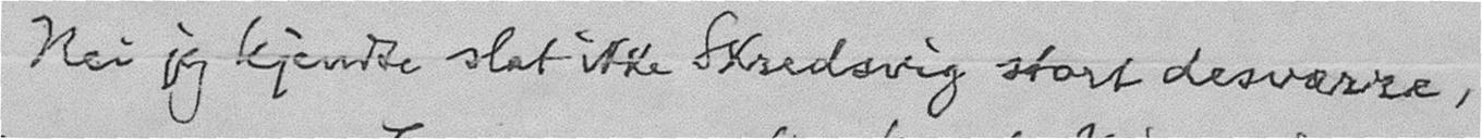Nei jeg kjendte slet ikke Skredsvig stort desværre,
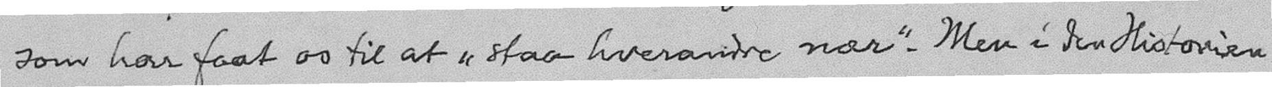som har faat os til at "staa hverandre nær". Men i den Historien
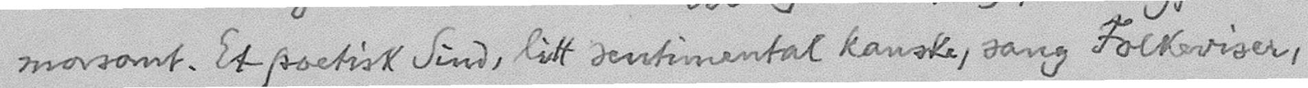morsomt. Et poetisk Sind, litt sentimental kanske, sang Folkeviser,
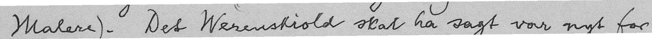Malere). Det Werenskiold skal ha sagt var nyt for
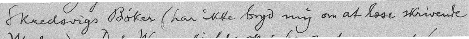Skredsvigs Bøker (har ikke bryd mig om at læse skrivende
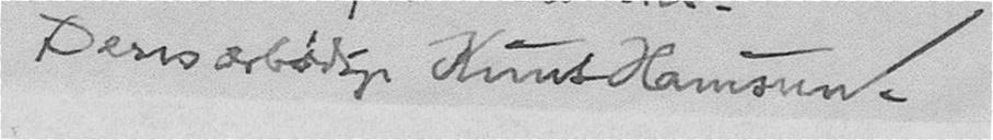Deres ærbødige Knut Hamsun.
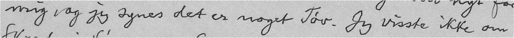mig og jeg synes det er noget Tøv. Jeg visste ikke om
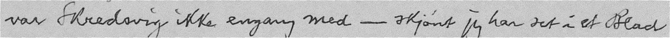var Skredsvig ikke engang med - skjønt jeg har set i et Blad
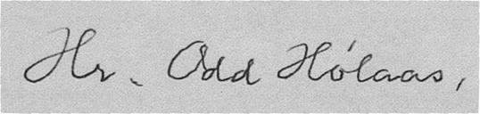Hr. Odd Hølaas,
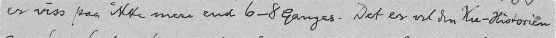er viss paa ikke mere end 6-8 Ganger. Det er vel den Ku-Historien
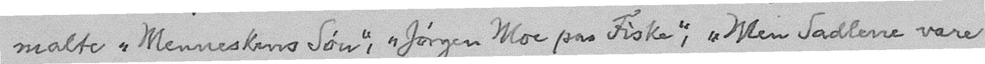malte "Menneskens Søn", "Jørgen Moe paa Fiske", "Men Sadlene vare
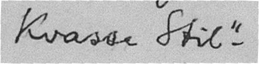kvasse Stil".
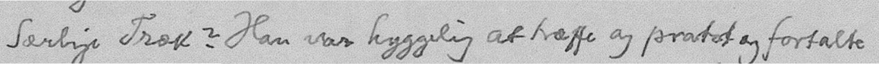Særlige Træk? Han var hyggelig at træffe og pratet og fortalte
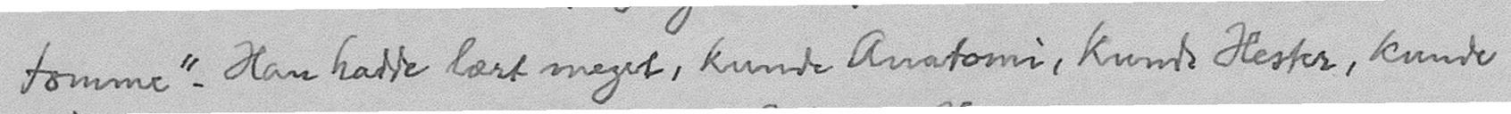tomme". Han hadde lært meget, kunde Anatomi, kunde Hester, kunde
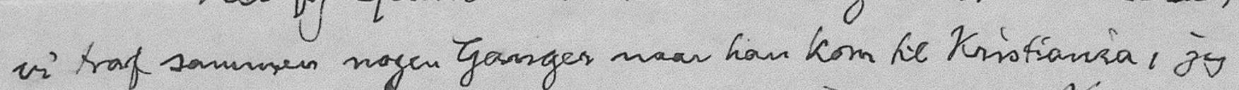vi traf sammen nogen Ganger naar han kom til Kristiania, jeg
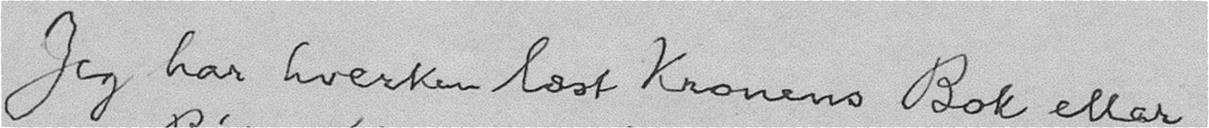Jeg har hverken læst Kronens Bok eller
